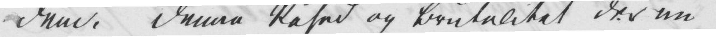dem. Denne Råhed og Brutalitet der nu
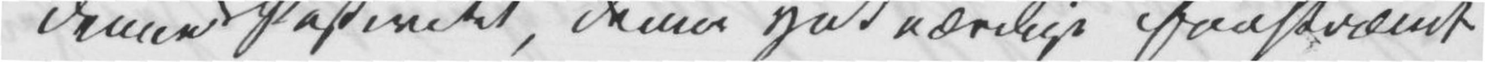denne Pasivitet, denne ynkværdige forskræmte
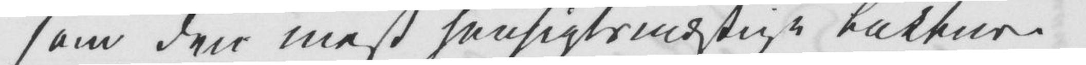som den mest hensigtsmæssige Lekture
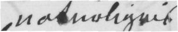naturligvis
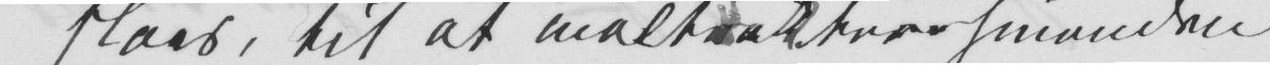slåes, til at maltraktere hinanden
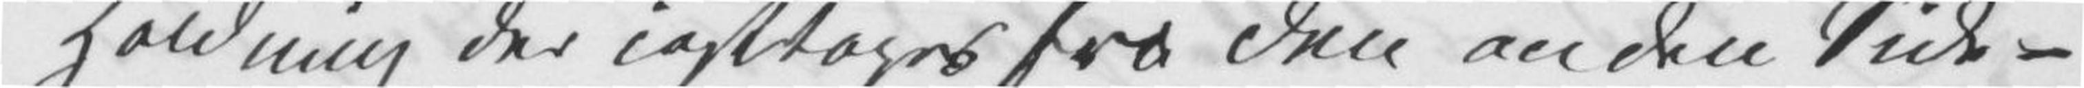Holdning der iagttages fra den anden Side –
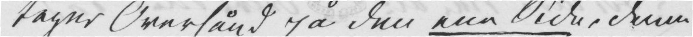tager Overhånd på den ene Side, denne
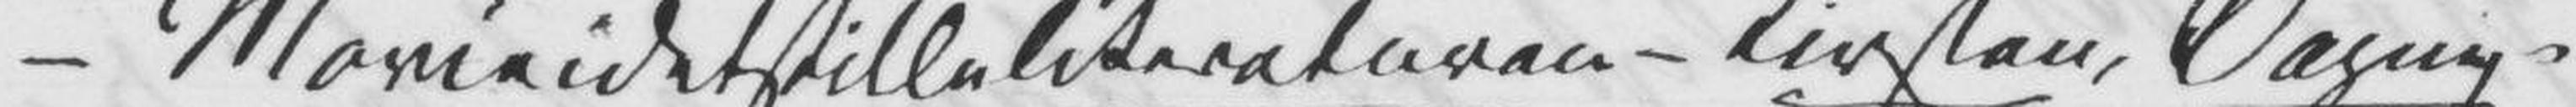Mariaidetstilleliteraturen – Kirsten, Dagny,
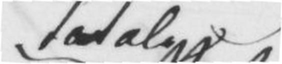fatale
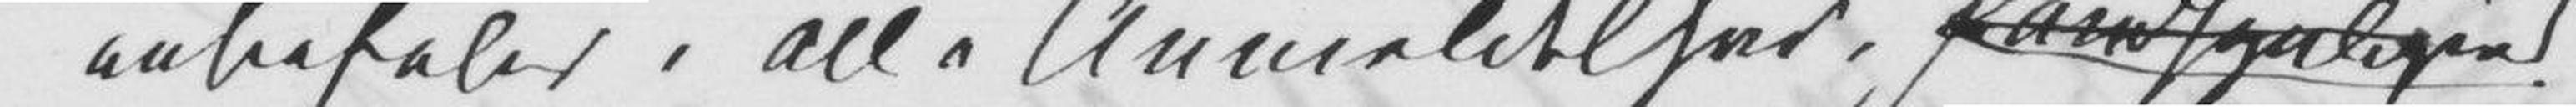anbefales i alle Anmeldelser, sandsynligvis
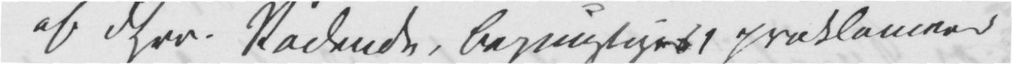af dHrr. Rådende, begunstiges, proklameres
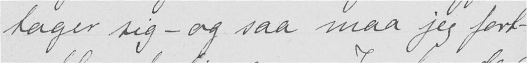tager sig- og saa maa jeg fort-
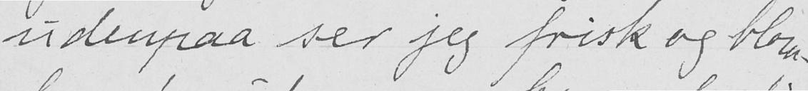udenpaa ser jeg frisk og blom-
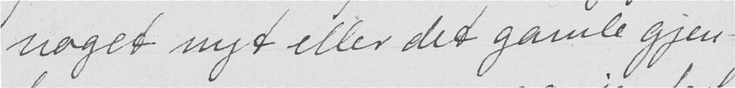noget nyt eller det gamle gjen-
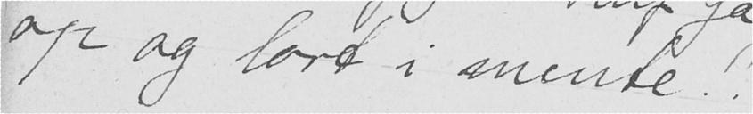op og lort i mente!!
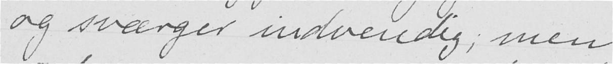og sværger indvendig; men
